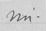nu!
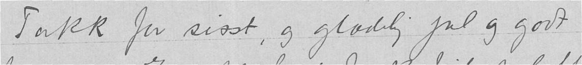Takk for sisst, og glædelig jul og godt
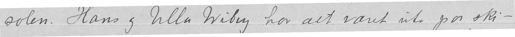solen. Hans og Ulla Wiberg har alt været ute paa ski -
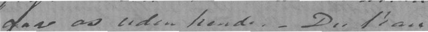gaae os uden hende. - Du kan
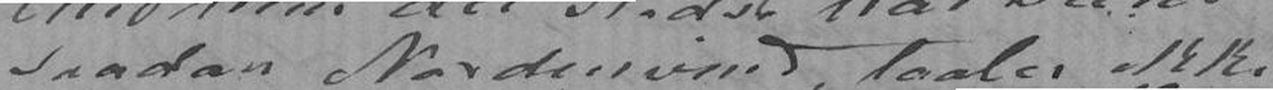saadan Nordenvind, taaler ikke
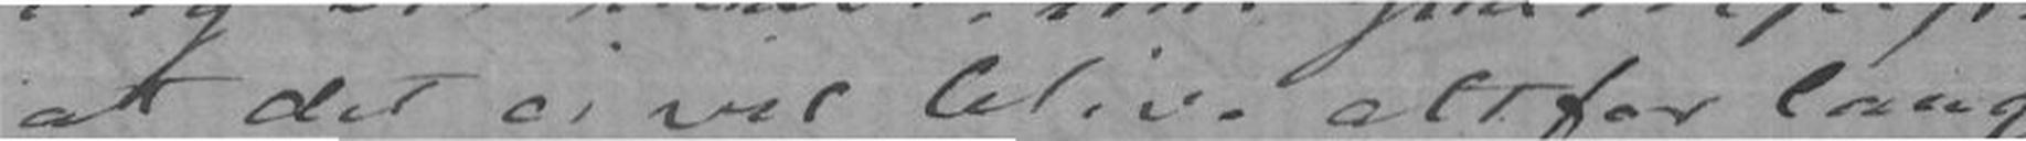at det ei vil blive altfor lang
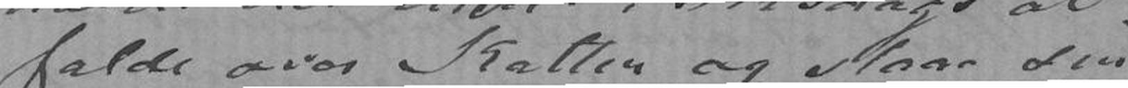falde over Katten og slaae sin
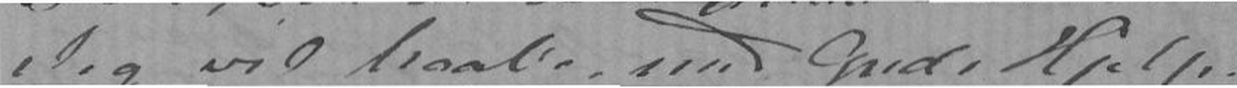Jeg vil haabe med Guds Hjelp
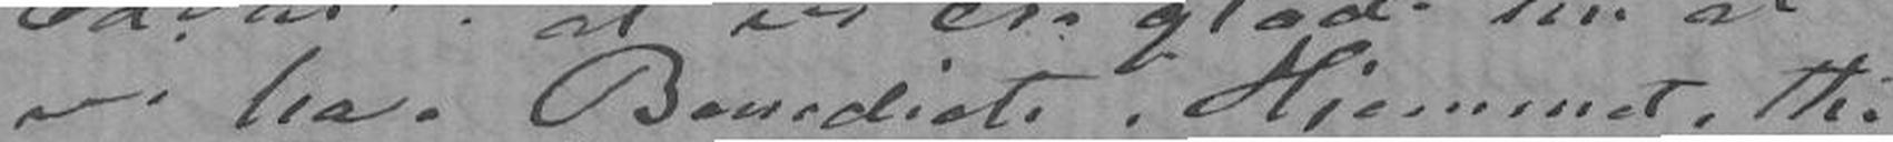vi har Benedicte i Hjemmet, thi
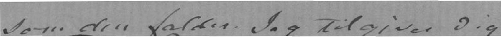som den falder. Jeg tilgiver Dig
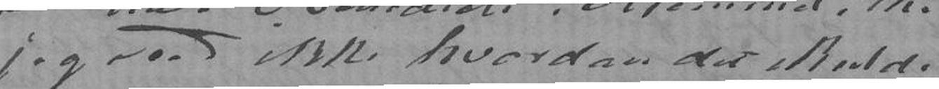jeg veed ikke hvordan det skulde
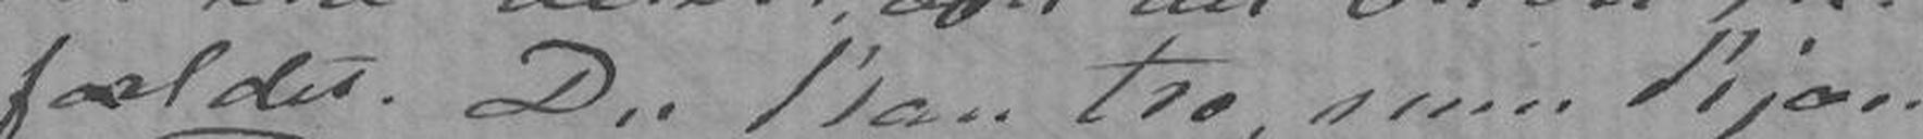fældet. Du kan tro, min kjære
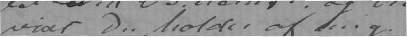vist Du holder af mig.
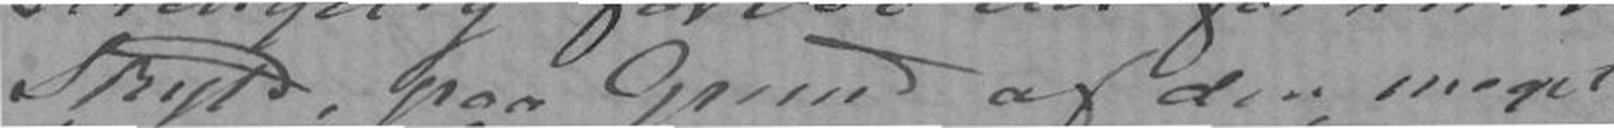Skyld, paa Grund af den meget
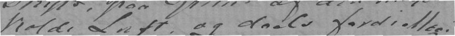kolde Luft, og deels fordi Moer
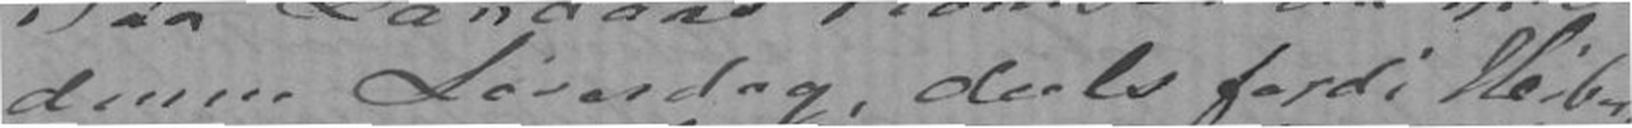denne Løverdag, deels fordi Heiberg
